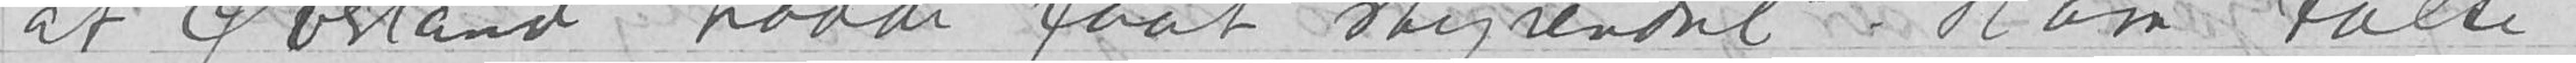at Øverland hadde faat stipendiet. Han talte
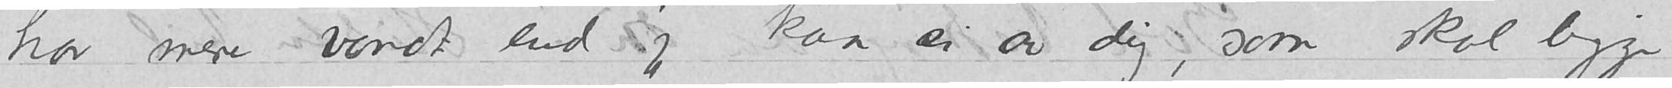har mere vondt end jeg kan si av dig, som skal ligge
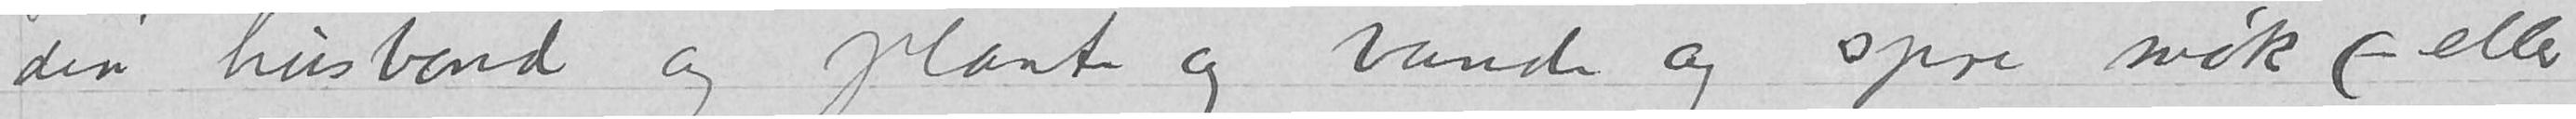din husbond og plante og vande og spre møk (– eller
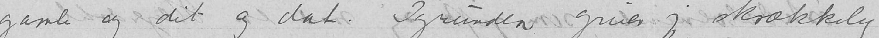gamle og dit og dat. Igrunden gruer jeg skrækkelig
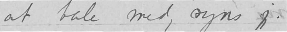at tale med, syns jeg.
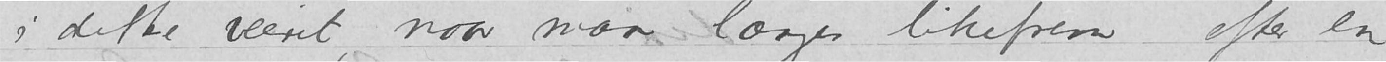i dette veiret, naar man længes likefrem efter en
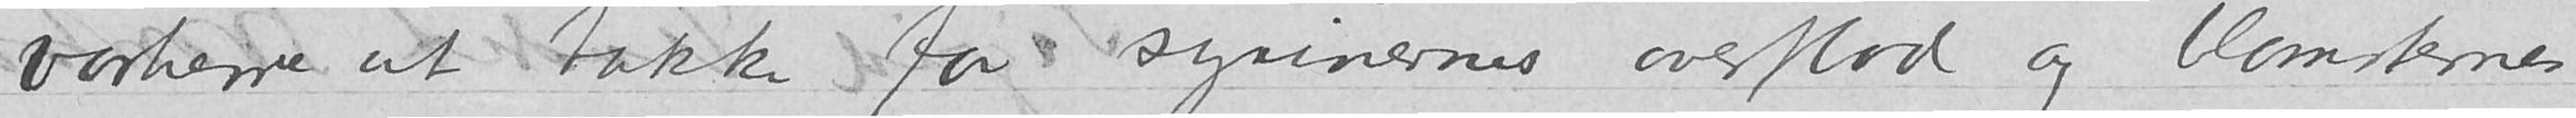vorherre at takke for syrinernes overflod og blomsternes
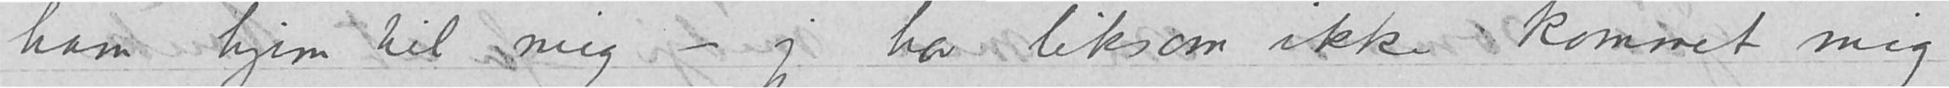ham hjem til mig – jeg har liksom ikke kommet mig
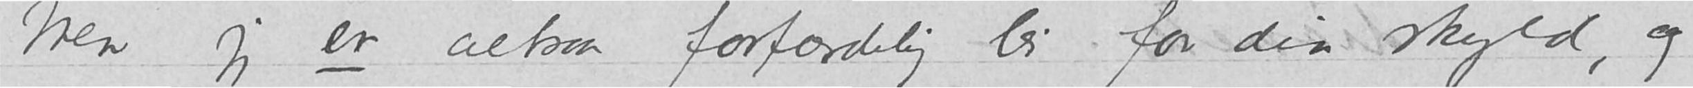Men jeg er altsaa forfærdelig lei for din skyld, og
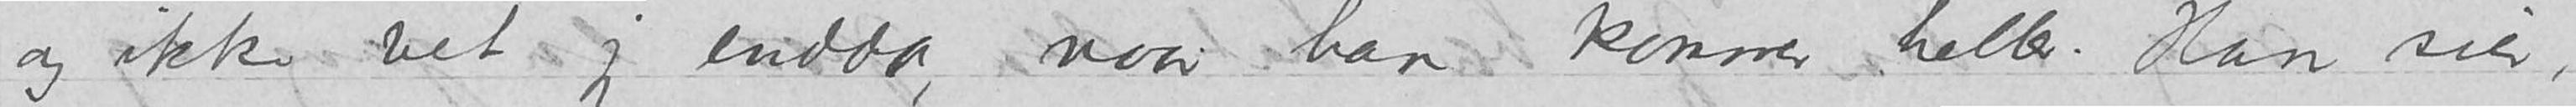og ikke vet jeg endda, naar han kommer heller. Han sier,
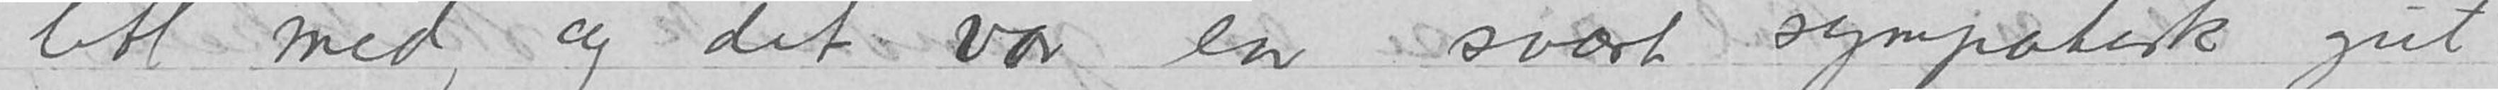litt med, og det var en svært sympatisk gut
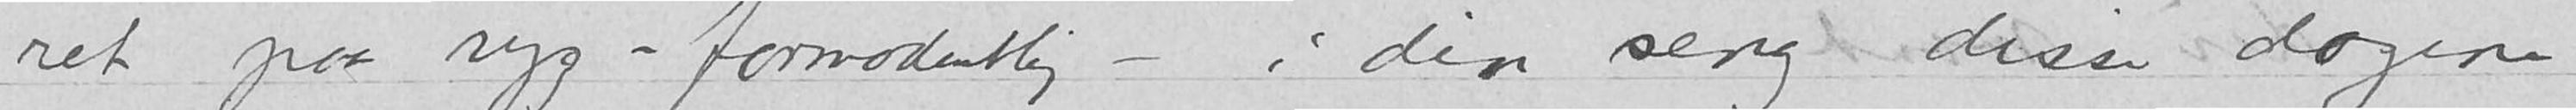ret paa ryg – formodentlig – i din seng disse dagene
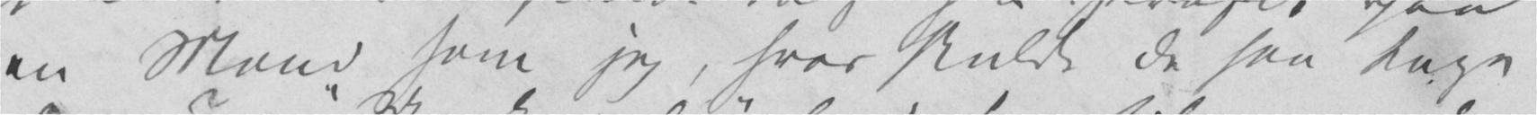en Mand som jeg, hvor skulde de saa tage
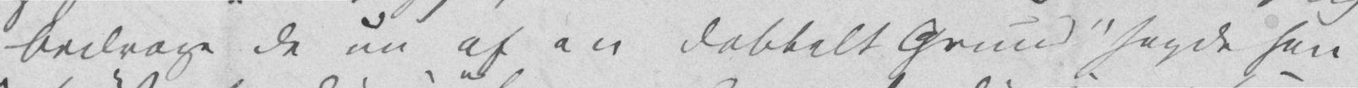bedrage de nu af en dobbelt Grund» sagde han
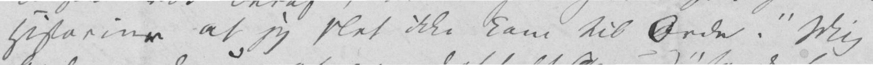Historier at jeg slet ikke kom til Orde. «Mig
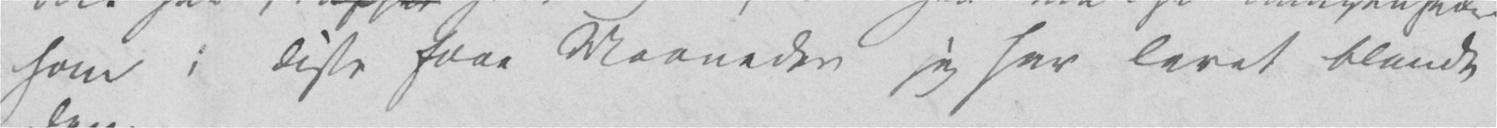som i disse faae Maaneder jeg har levet blandt
